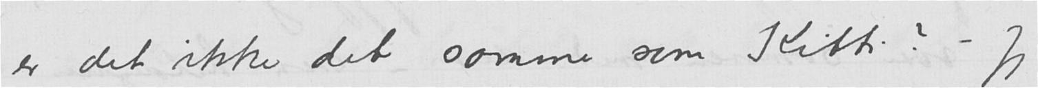er det ikke det samme som Kitti? - Jeg
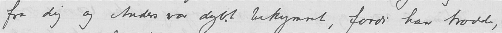fra dig og Anders var dybt bekymret, fordi han trodde,
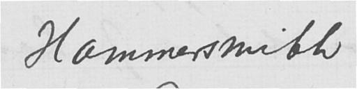Hammersmith
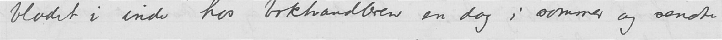bladet i inde hos bokhandleren en dag i sommer og sendte
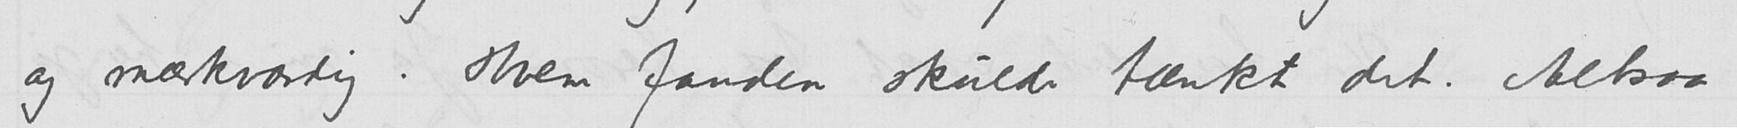og mærkværdig. Hvem fanden skulde tænkt det. Altsaa
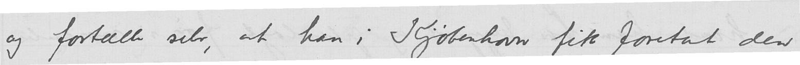og fortælle selv, at han i Kjøbenhavn fik foretat den
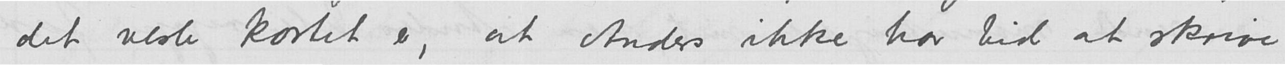det vesle kortet er, at Anders ikke har tid at skrive
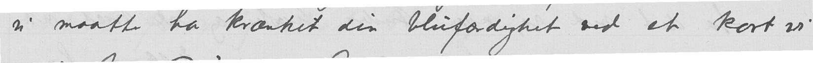vi maatte ha krænket din blufærdighet ved et kort vi
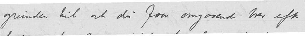grunden til at du faar omgaaende brev efter
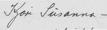Kjære Susanna -
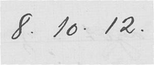8.10.12.
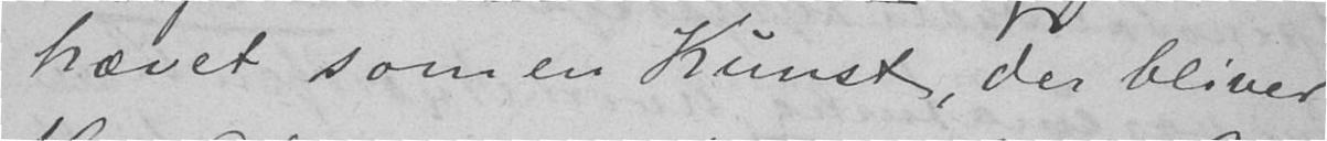hævet som en Kunst, der bliver
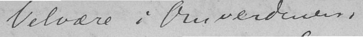Velvære i Omverdenen.
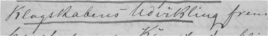Klogskabens Udvikling frem
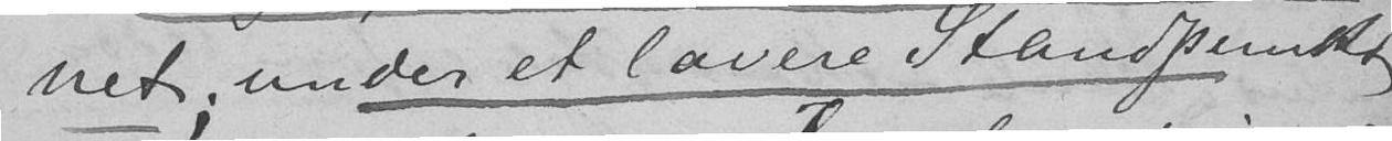net; under et lavere Standpunkt
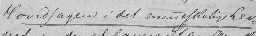Hovedsagen i det menneskelige Lev
